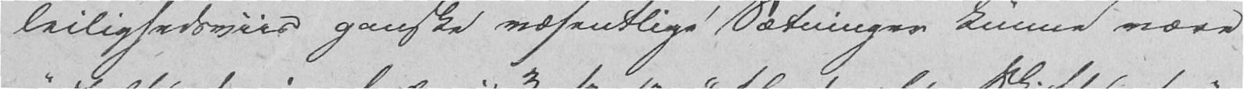leilighedsviis ganske væsentlige Sætninger kunne være
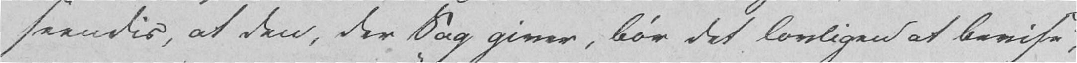seendis, at den, der Sag giver, bør det lovligen at bevise",
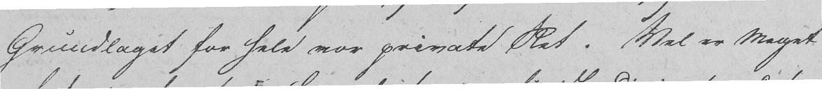Grundlaget for hele vor private Ret. Vel er meget
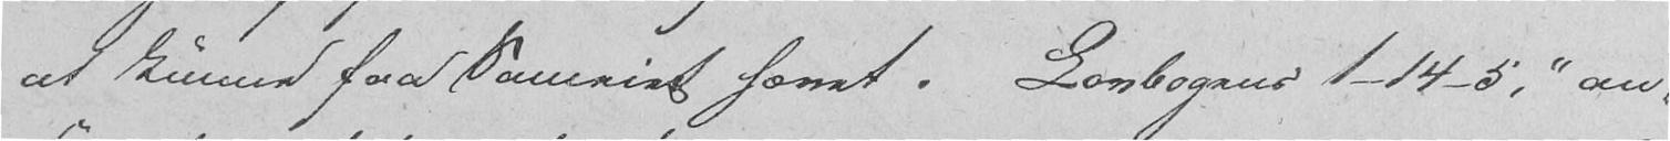at kunne faa Sameiet hævet. Lovbogens 1-14-5, "an-
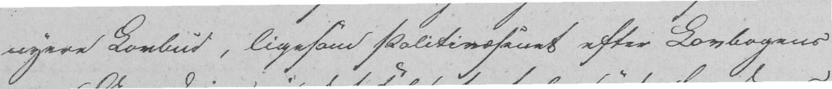nyere Lovbud, ligesom Politivæsenet efter Lovbogens
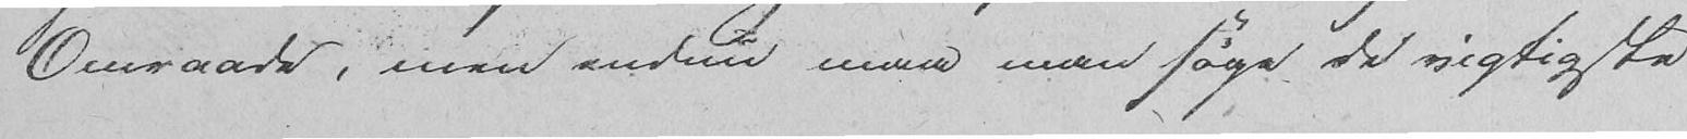Omraade, men endnu maa man søge de vigtigste
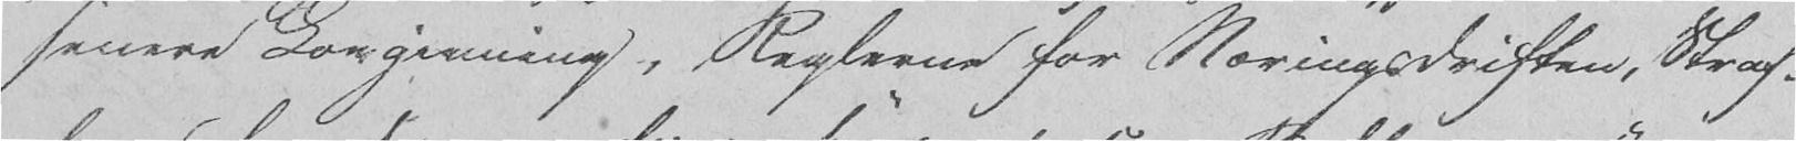senere Lovgivning, Reglerne for Næringsdriften, Straf-
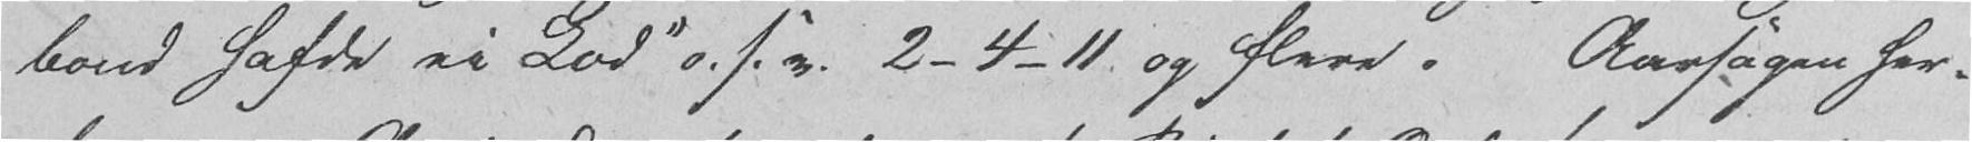bond hafde ei Lod" o. s. v. 2-4-11 og flere. Aarsagen her-
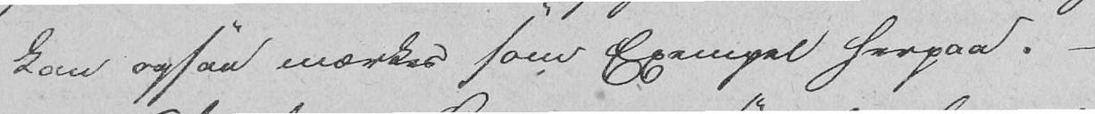kan ogsaa mærkes som Exempel herpaa. -
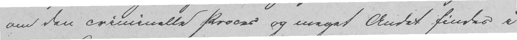om den criminelle Proces og meget Andet findes i
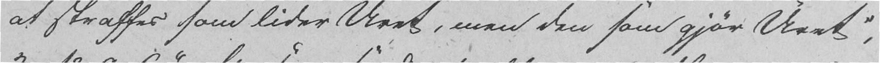at straffes som lider Uret, men den som gjør Uret",
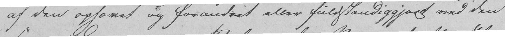af den ophævet og forandret eller fuldstændiggjort ved den
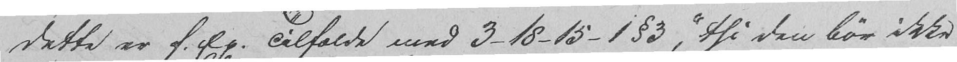dette er f. Ex. Tilfælde med 3-18-15-1 § 3, "thi den bør ikke
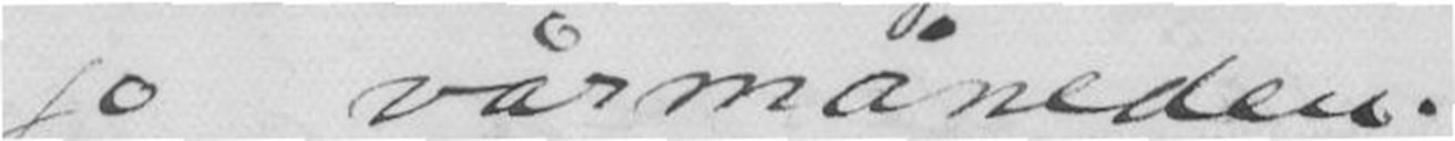jo vårmåneden.
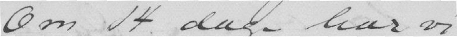Om 14 dage har vi
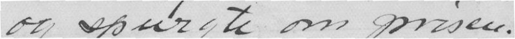og spurgte om prisen.
